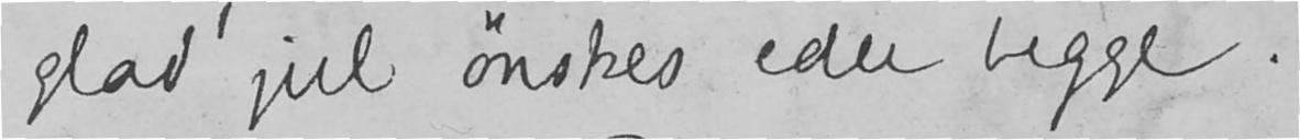glad jul ønskes eder begge.
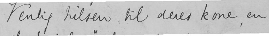Venlig hilsen til deres kone, en
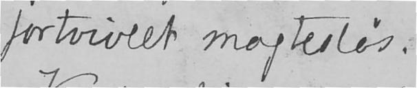fortvivlet magtesløs.
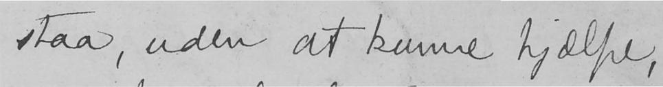staa, uden at kunne hjælpe,
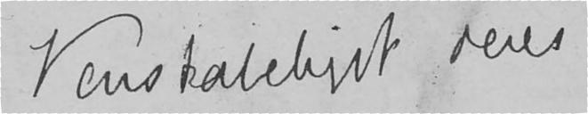Venskabeligst deres
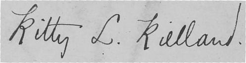Kitty L. Kielland.
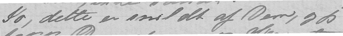Jo, dette er snildt af Dem, og jeg
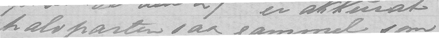halvparten saa gammel som
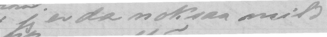jeg er da noksaa mild
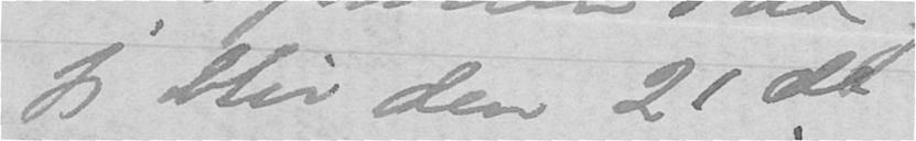jeg blir den 21de.
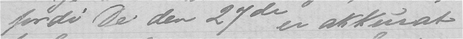fordi De den 27de er akkurat
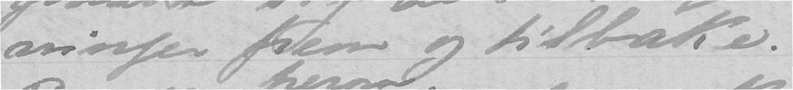ninger frem og tilbake.
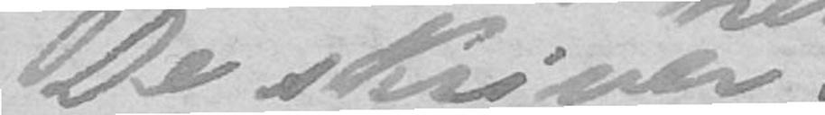De skriver
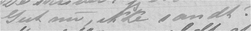Gut nu, ikke sandt?
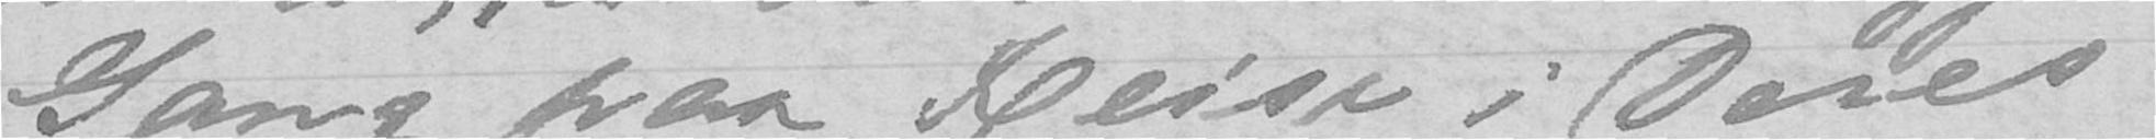Gang paa Reise i Deres
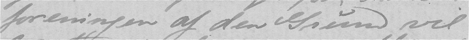foreningen af den Grund vil
